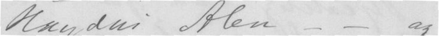Hayden's Alen - - og
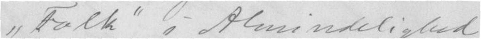Folk i Almindelighed,
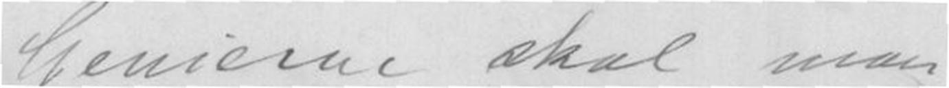Genierne skal man
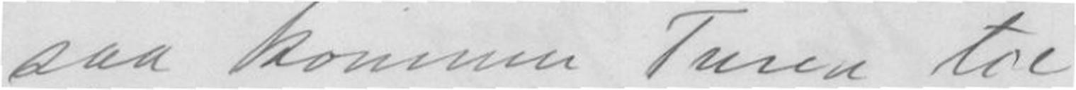saa kommer Turen til
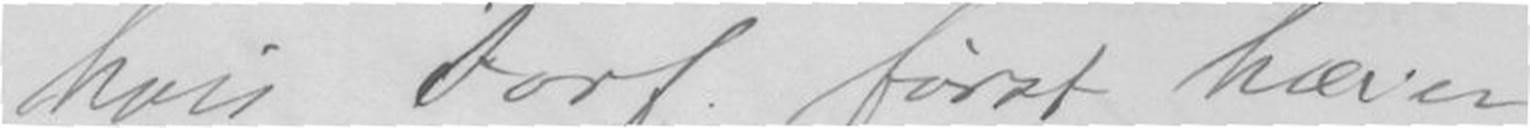hvis Forf. først hæver
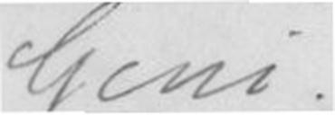Geni
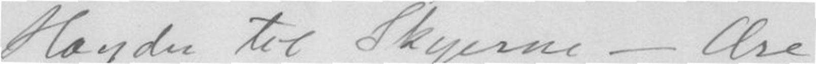Haydn. til Skyerne - Ære
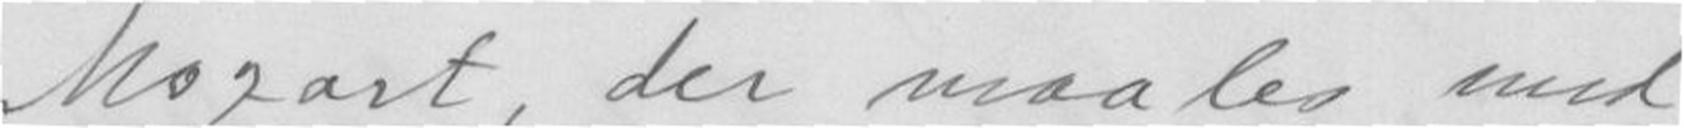Mozart, der maales med
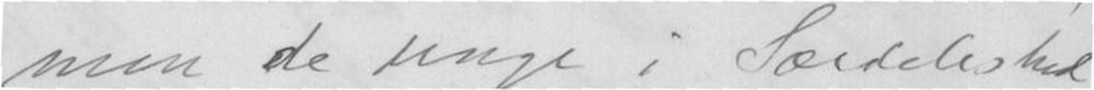men de unge i Særdeleshed
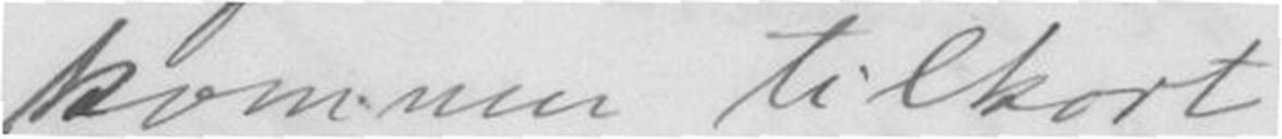kommer tilkort
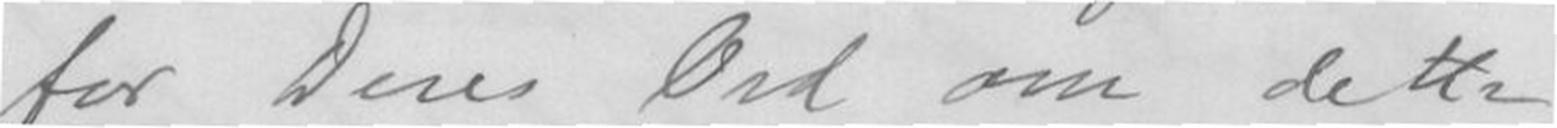for Deres Ord om dette
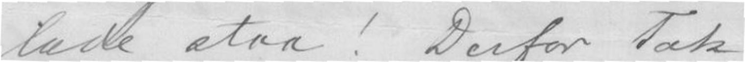lade staa! Derfor Tak
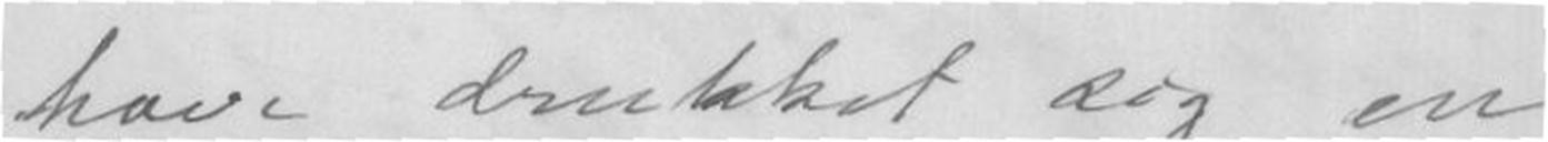have drukket sig en
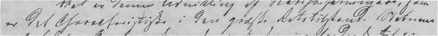er det charakteristiske i den græske Retstilstand. Staternes
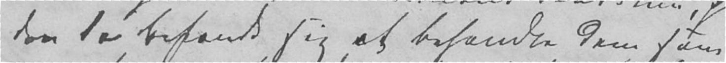den da befandt sig at behandle dem som
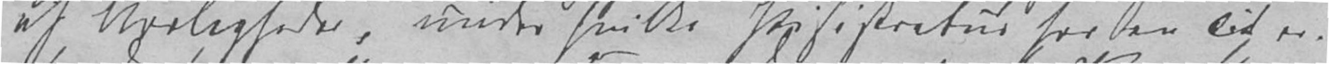af Uroligheder, under hvilke Peisistratus for en Tid er-
